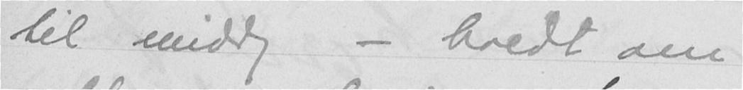til middag - holdt om
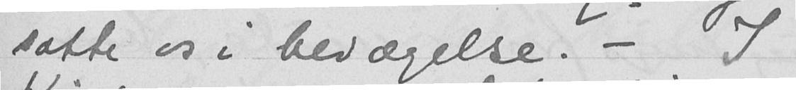satte os i bevægelse. - I
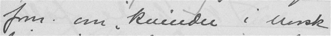forn. om "kvinder i norsk
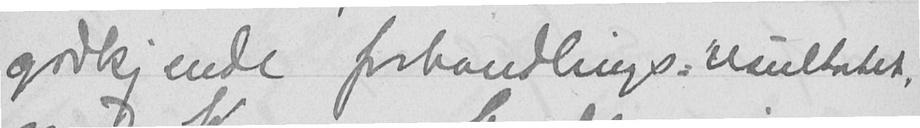godkjende forhandlings-resultatet,
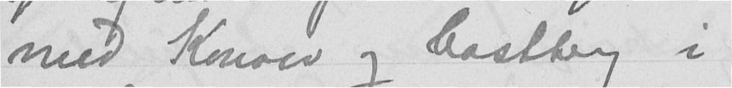med Konow og Castberg i
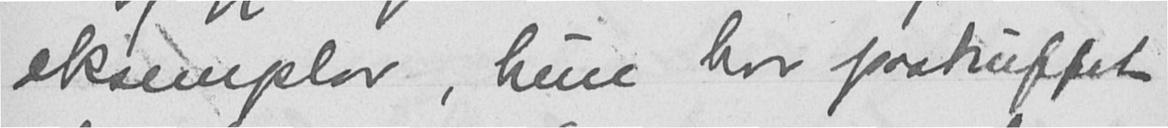eksemplar, hun har paatruffet
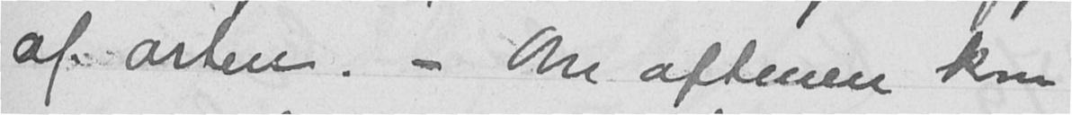af arten. - Om aftenen kom
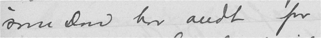som Don har ondt for
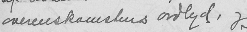overenskomstens ordlyd, og
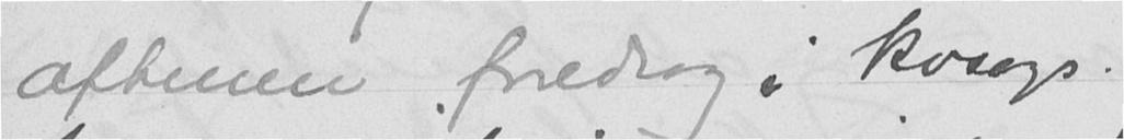aftenen foredrag i kvsags.
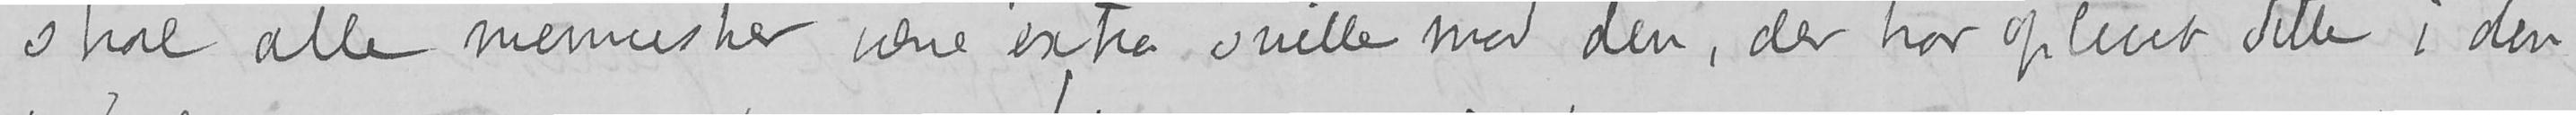skal alle mennesker være extra snille mod den, der har oplevet dette i den
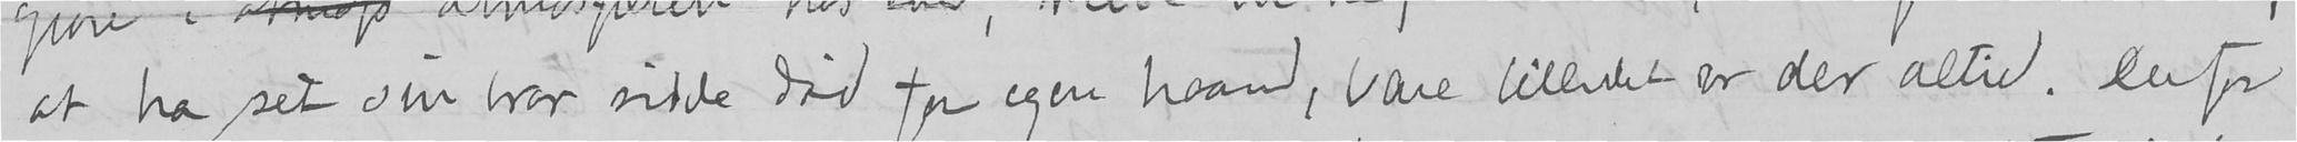at ha set sin bror sidde død for egen haand, bare billedet er der altid. Derfor
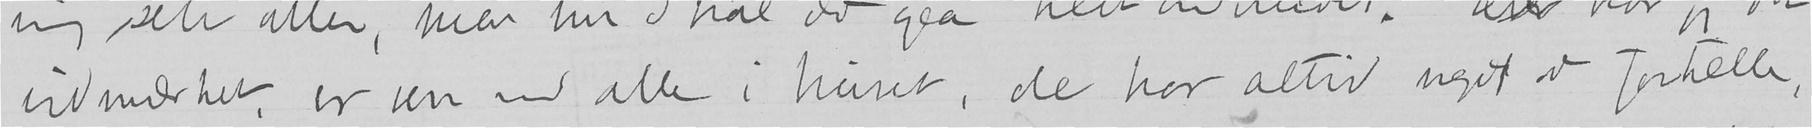udmærket, er ven med alle i huset, de har altid noget at fortelle,
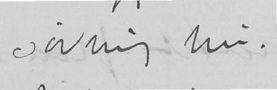søvnig nu.
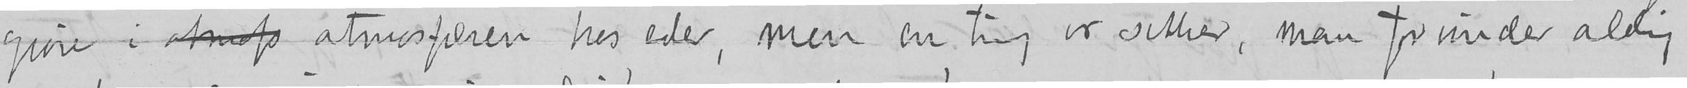gjøre i atmofs atmosfæren hos eder, men en ting er sikker, man forunder aldrig
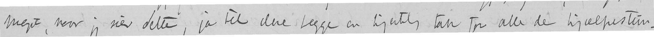meget, naar jeg sier dette, ja til dere begge en hjertelig tak for alle de hjælpestun-
